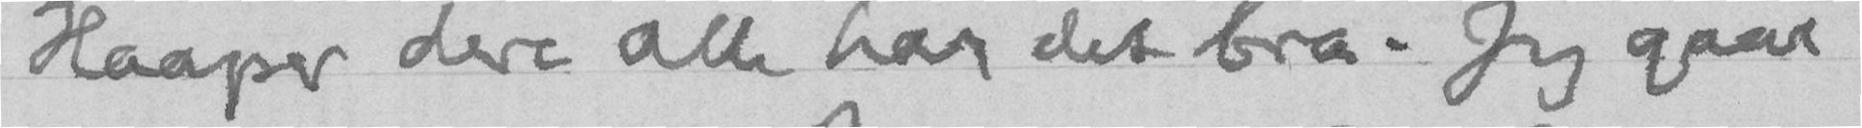Haaper dere alle har det bra. Jeg gaar
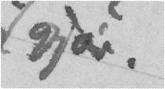gjør.
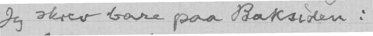Jeg skrev bare paa Baksiden:
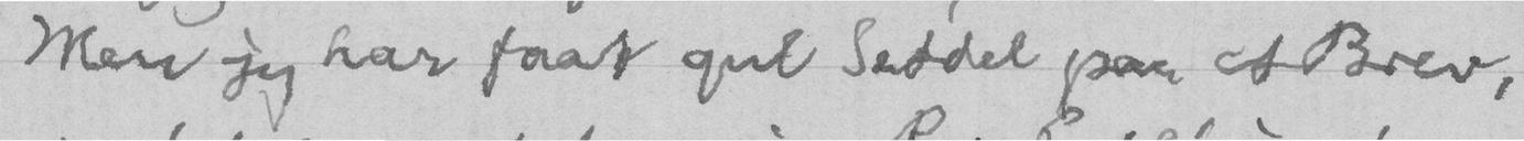Men jeg har faat gul Seddel paa et Brev,
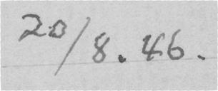20/8. 46.
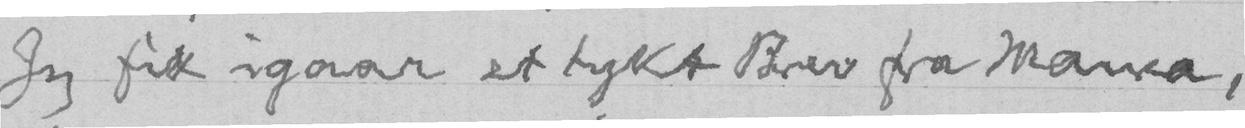Jeg fik igaar et tykt Brev fra Mama,
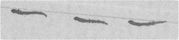- - -
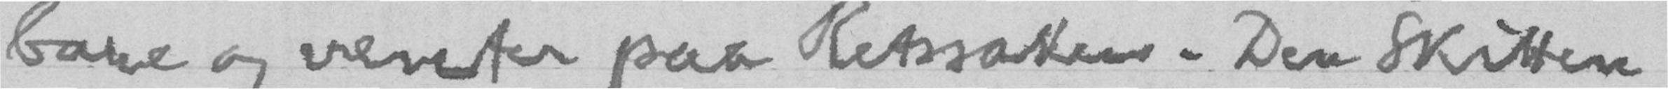bare og venter paa Retssaken. Den Skitten
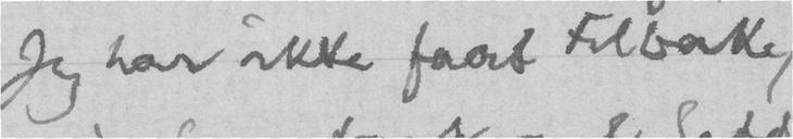Jeg har ikke faat tilbake
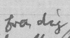fra dig
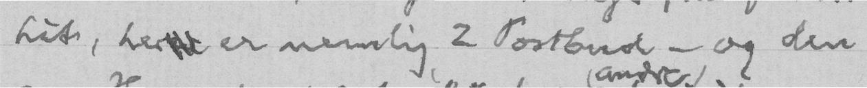hit, herer er nemlig 2 Postbud - og den
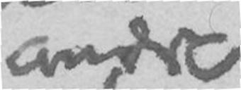andre
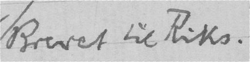Brevet til Riks.
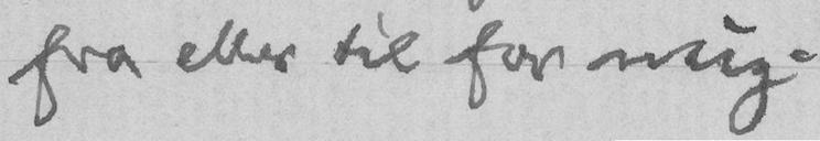fra eller til for mig.
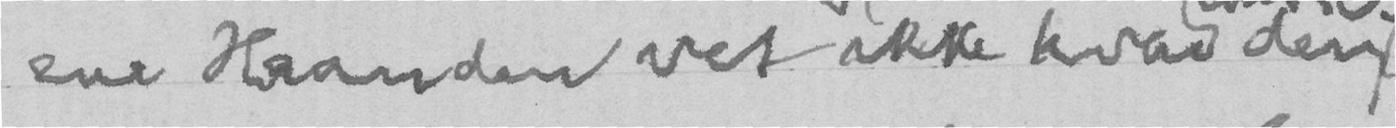ene Haanden vet ikke hvad den
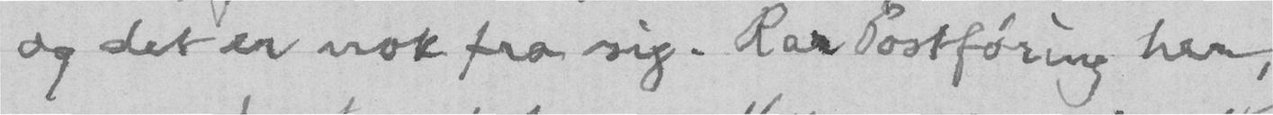og det er nok fra dig. Rar Postføring her,
